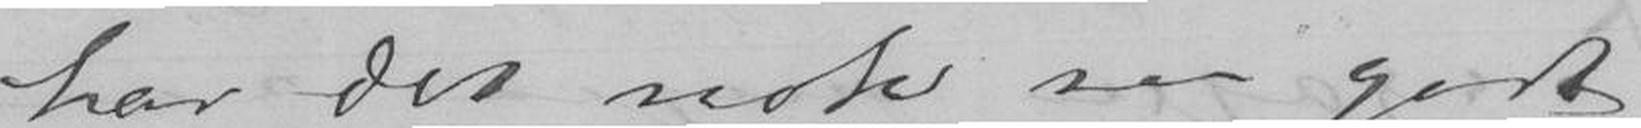har det nok saa godt
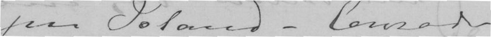paa Island - Conradi
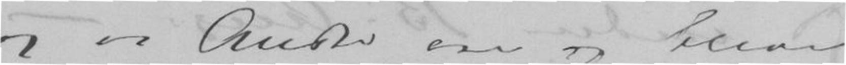og vi Andre ere og bliver
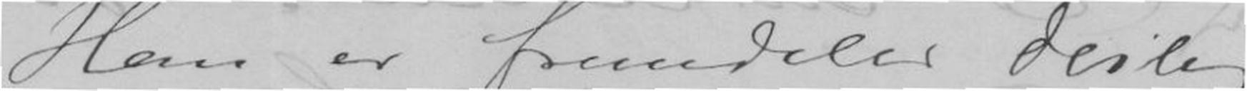Han er fremdeles deilig
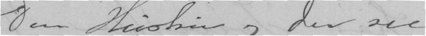Din Hustru og du selv
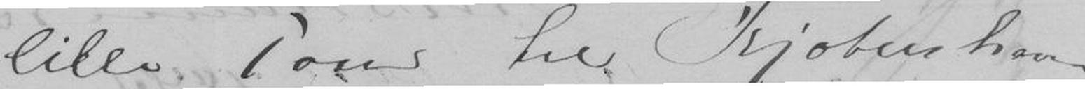lille Tour til Kjøbenhavn
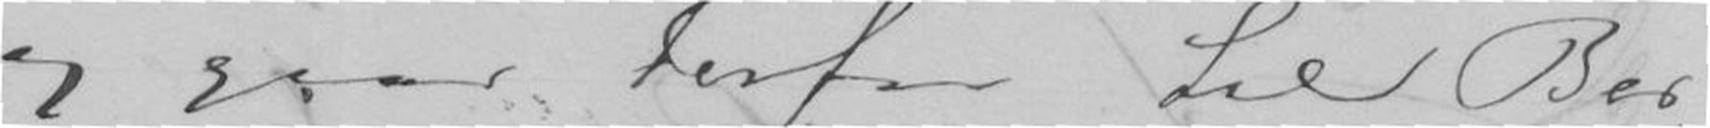og gaar derfra til Ber-
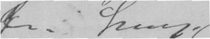fra heng.
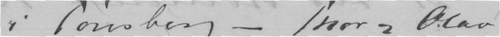i Tønsberg - Thor og Olav
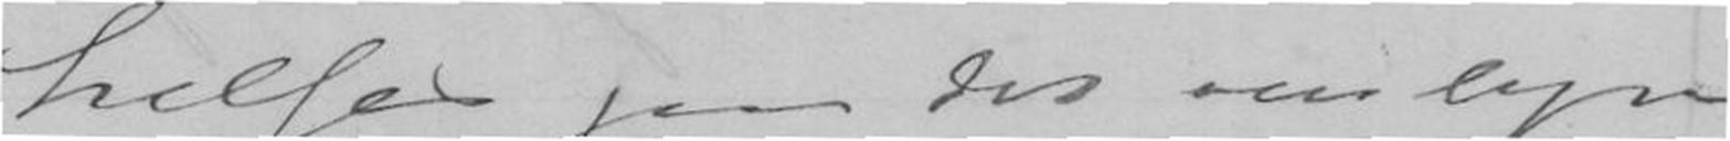hilses paa det venligte
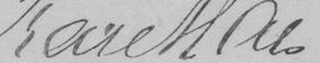Karl Hals
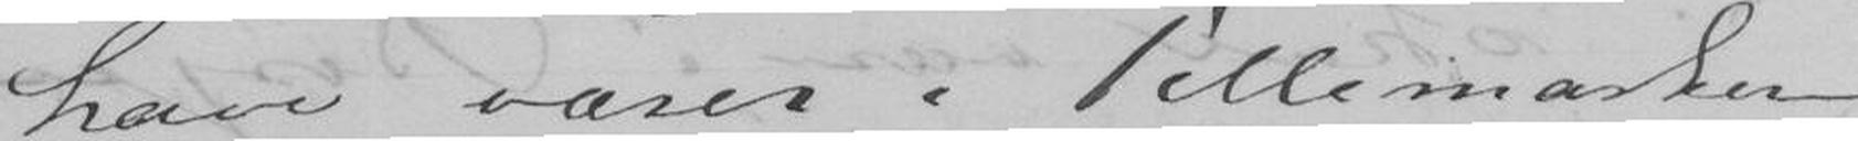have været i Tellemarken
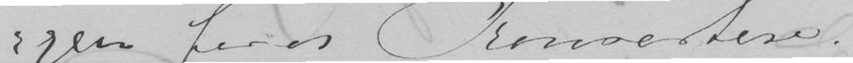gen forat Konsertere.
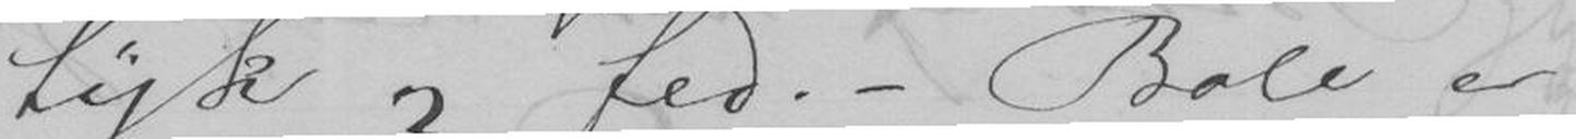tyk og fed. - Bole er
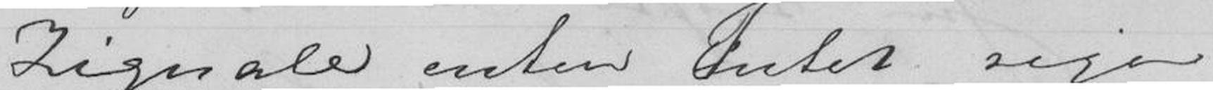Zignale enten Intet siger
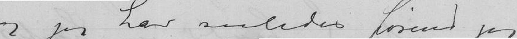og jeg har saaledes førend jeg
